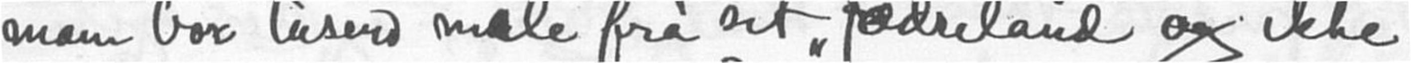man bor tusend mile fra sit "fædreland" og ikke
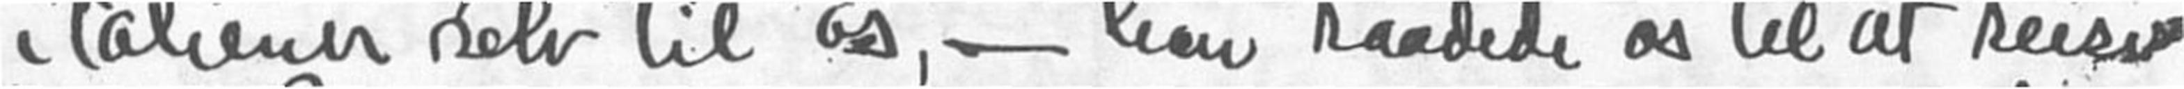italiener selv til os, - han raadede os til at reise
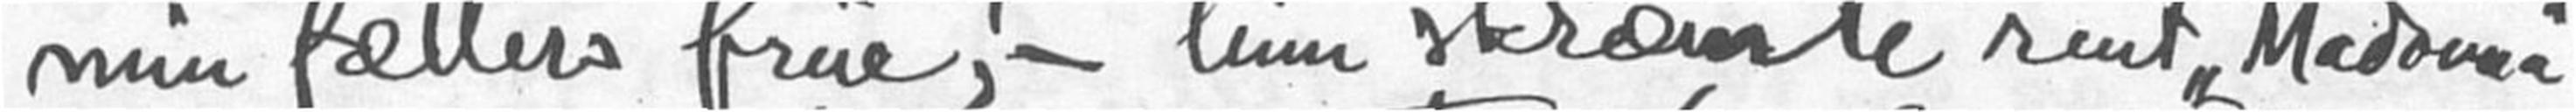min fætters frue, - hun skræmte rent "Madonna"
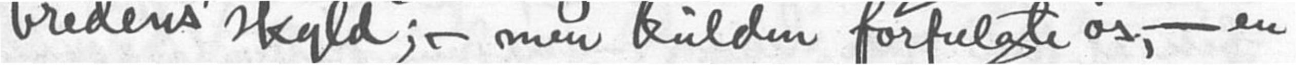bredens" skyld; - men kulden forfulgte os, - en
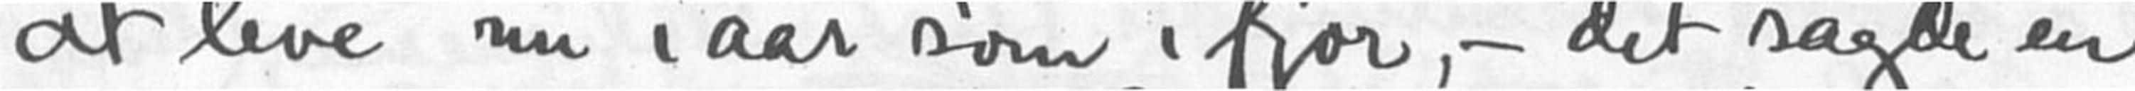at leve nu iaar som ifjor, - det sagde en
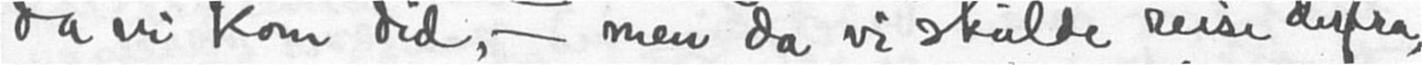da vi kom did, - men da vi skulde reise derfra
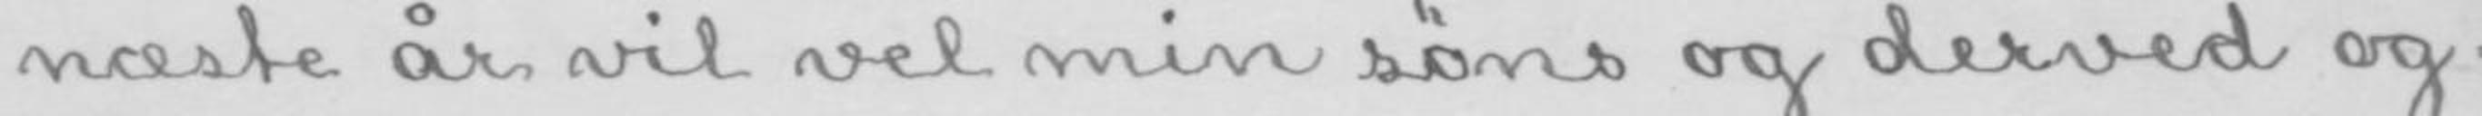næste år vil vel min søns og derved og-
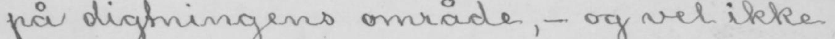på digtningens område,- og vel ikke
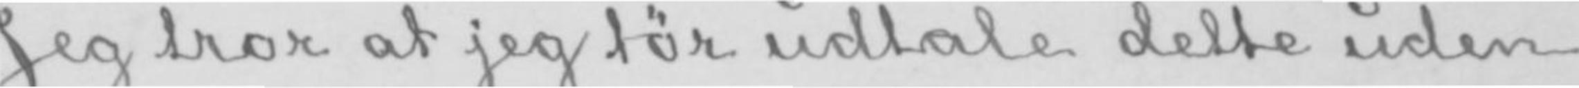Jeg tror at jeg tør udtale dette uden
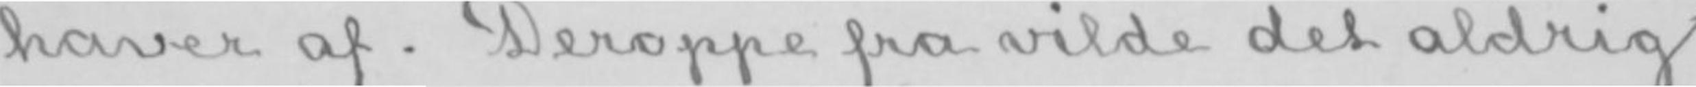haver af. Deroppe fra vilde det aldrig
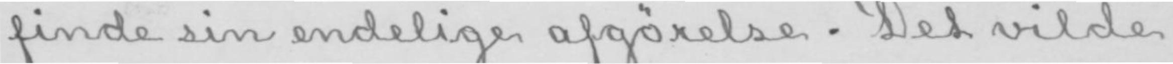finde sin endelige afgørelse. Det vilde
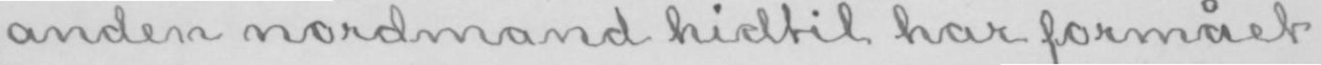anden nordmand hidtil har formået
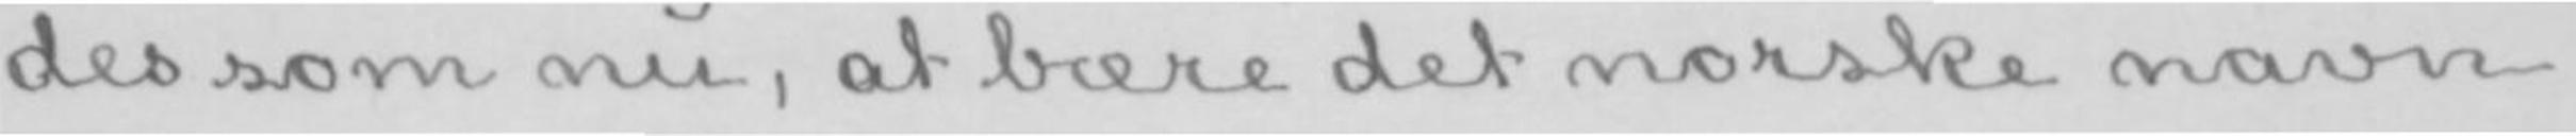des som nu, at bære det norske navn
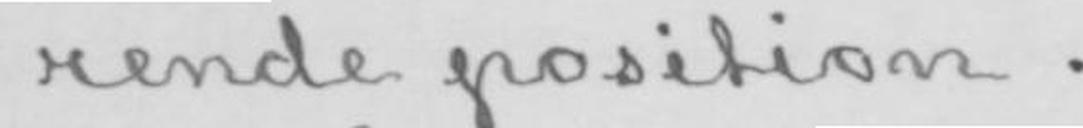rende position.
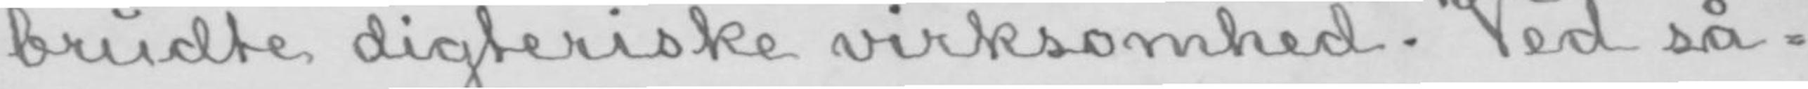brudte digteriske virksomhed. Ved så-
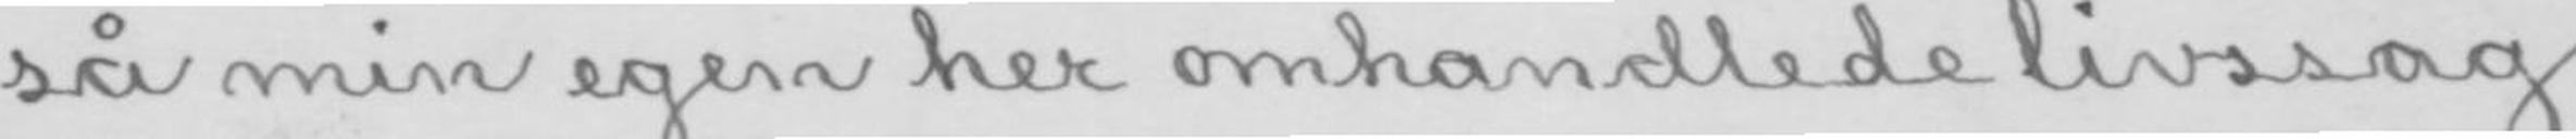så min egen her omhandlede livssag
